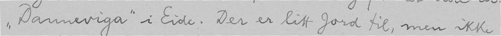"Danneviga" i Eide. Der er litt Jord til, men ikke
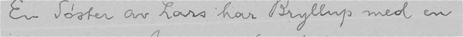En Søster av Lars har Bryllup med en
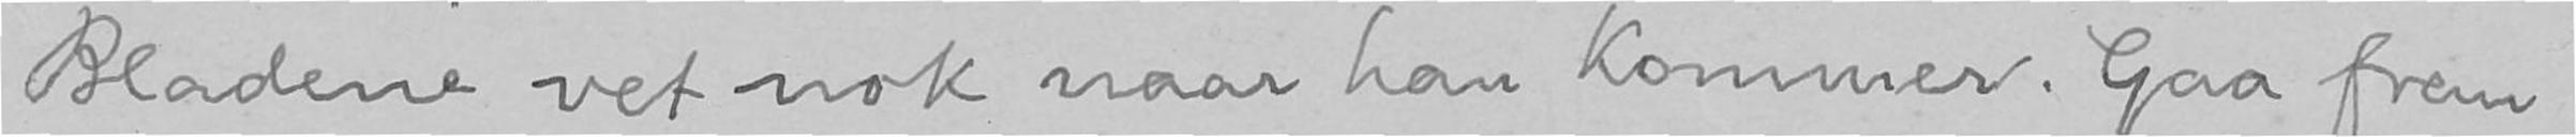Bladene vet nok naar han kommer. Gaa frem
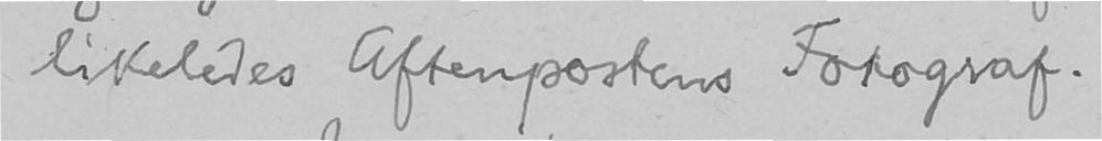likeledes Aftenpostens Fotograf.
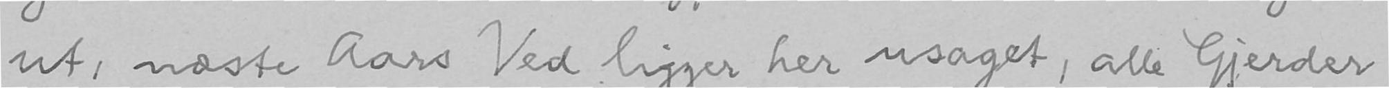ut, næste Aars Ved ligger her usaget, alle Gjerder
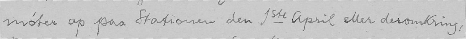møter op paa Stationen den 1ste April eller deromkring,
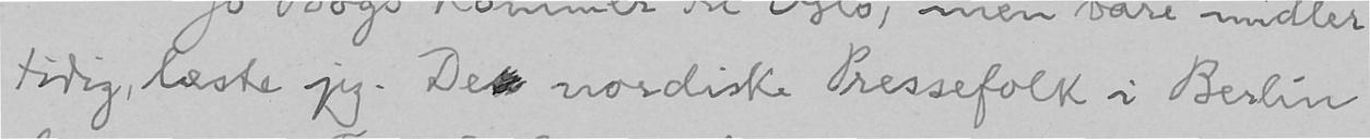tidig, læste jeg. Det nordiske Pressefolk i Berlin
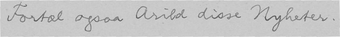Fortæl ogsaa Arild disse Nyheter.
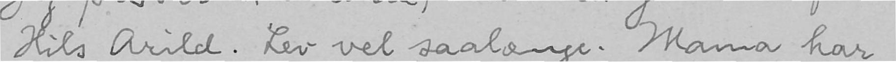Hils Arild. Lev vel saalænge. Mama har
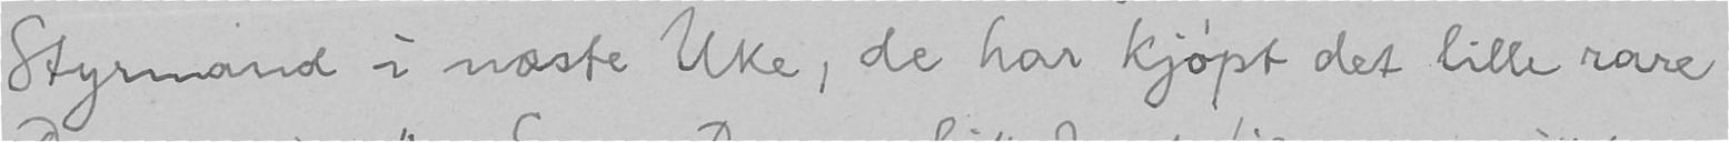Styrmand i næste Uke, de har kjøpt det lille rare
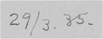29/3. 35.
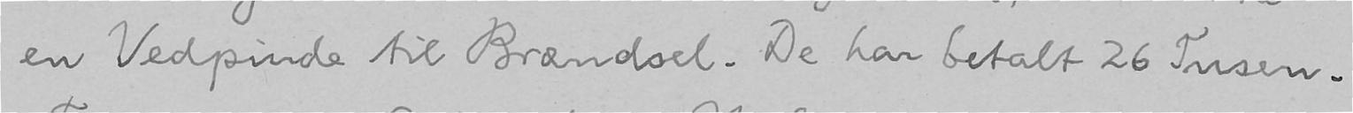en Vedpinde til Brændsel. De har betalt 26 Tusen.
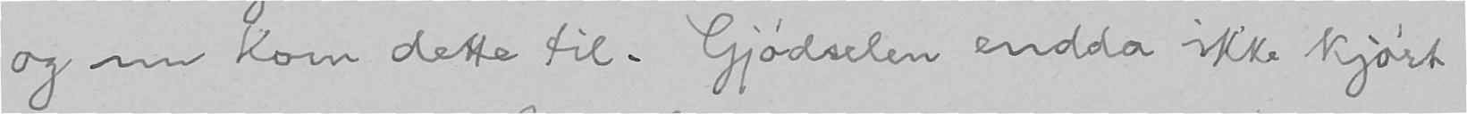og nu kom dette til. Gjødselen endda ikke kjørt
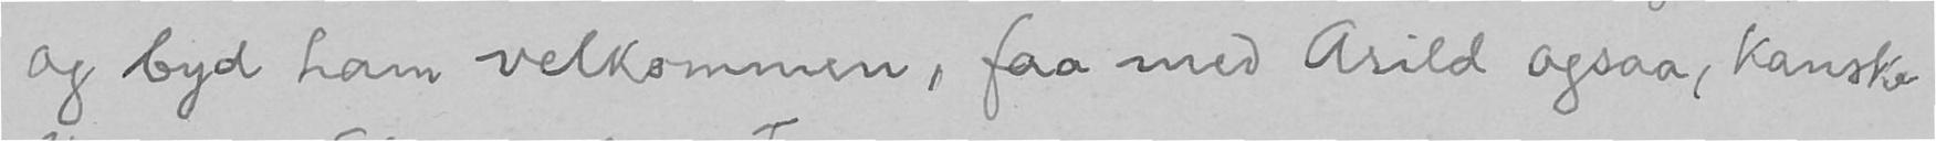og byd ham velkommen, faa med Arild ogsaa, kanske
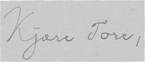Kjære Tore,
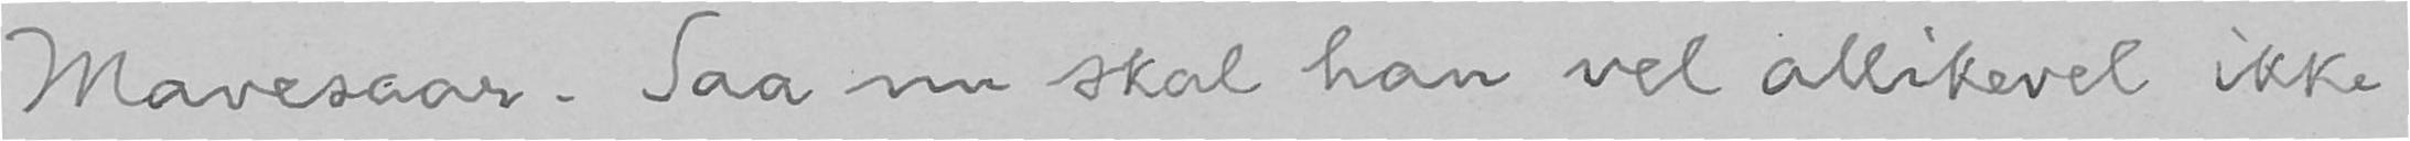Mavesaar. Saa nu skal han vel allikevel ikke
